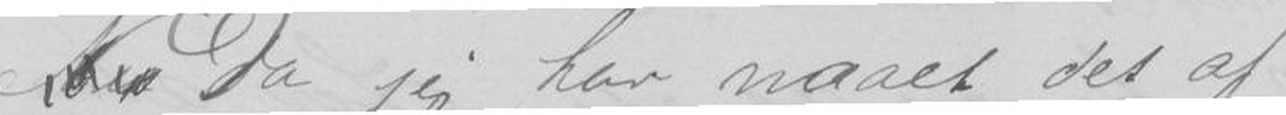Da jeg har naaet det af
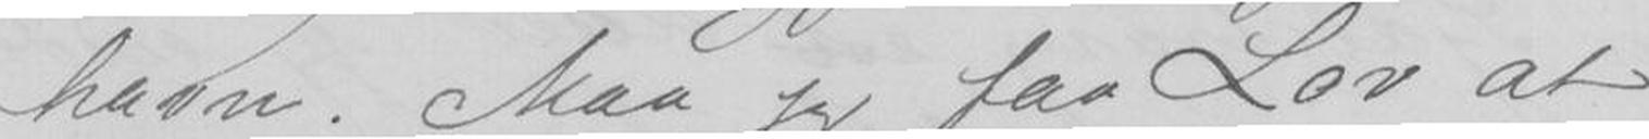havn. Kan jeg faa Lov at
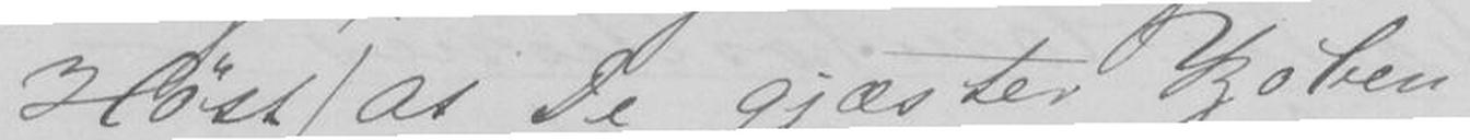Høst), At De gjæster Kjøben-
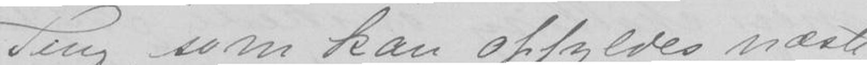Ting, som kan opfyldes næste
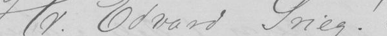Hr. Edvard Grieg!
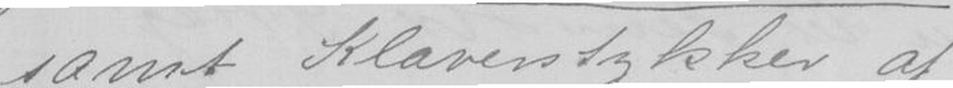samt Klaverstykker af
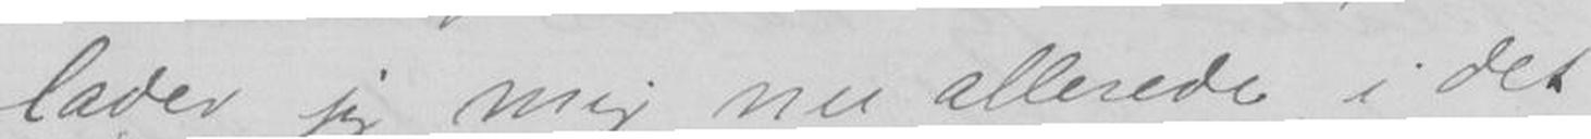lader jeg mig nu allerede, i det
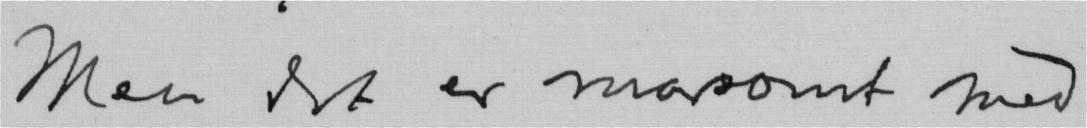Men det er morsomt med
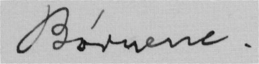Børnene.
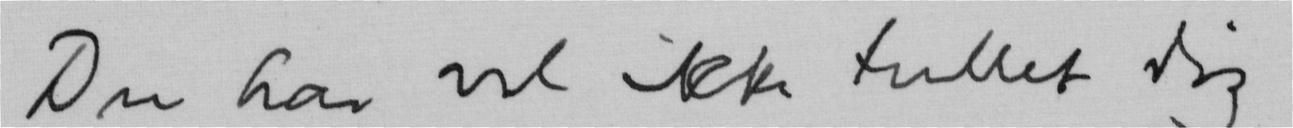Du har vel ikke tullet dig
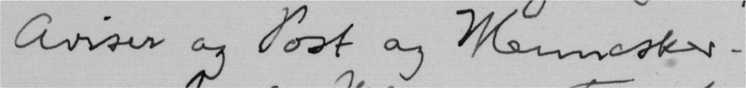Aviser og Post og Mennesker.
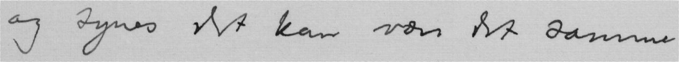og synes det kan være det samme
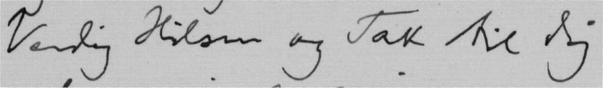Venlig Hilsen og Tak til dig
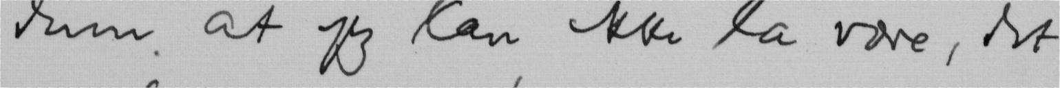dum at jeg kan ikke la være, det
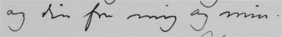og din fra mig og min.
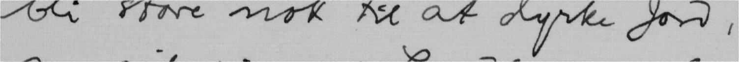bli store nok til at dyrke Jord,
